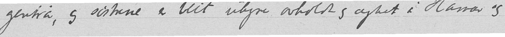gentia, og søstrene er blit uhyre avholdt og agtet i Hamar og
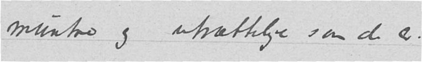muntre og utrættelige som de er.
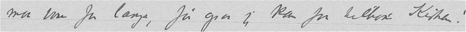maa vare for længe, før ogsaa jeg kan faa tilhøre Kirken!
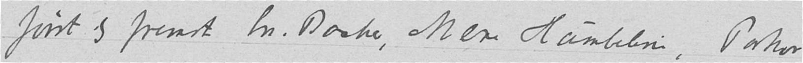først og fremst hr. Backer, Mere Humbeline, Pastor
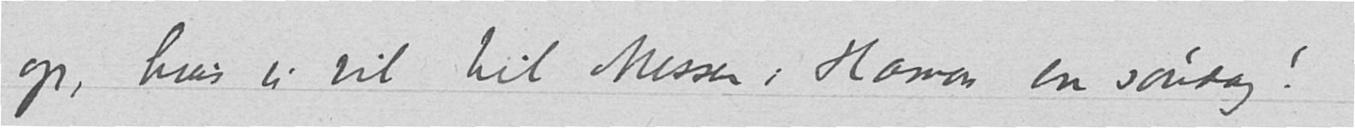op, hvis vi vil til Messen i Hamar en søndag!
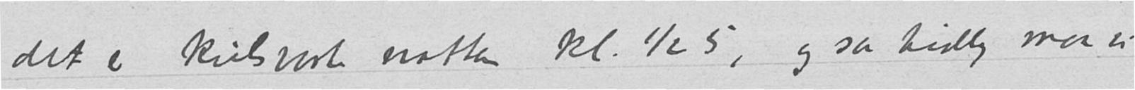det er kulsvarte natten kl. ½ 5, og saa tidlig maa vi
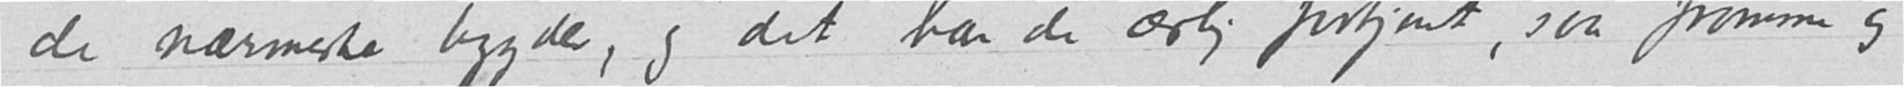de nærmeste bygder, og det har de ærlig fortjent, saa fromme og
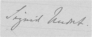Sigrid Undset.
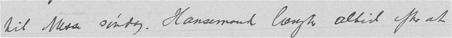til Messe søndag. Hansemand længter altid efter at
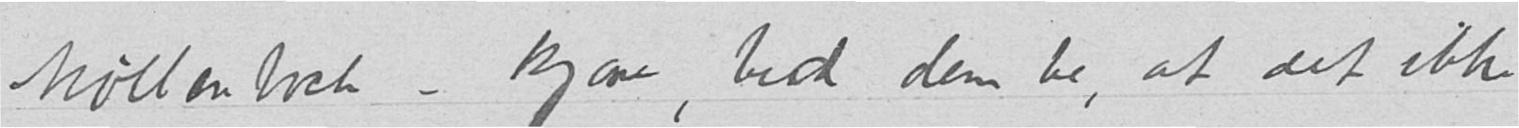Møllenbeck - kjære, bed dem be, at det ikke
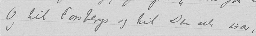Og til Forsbergs og til Dem selv især,
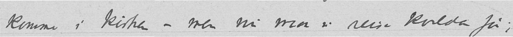komme i kirken – men nu maa vi reise kvelden før;
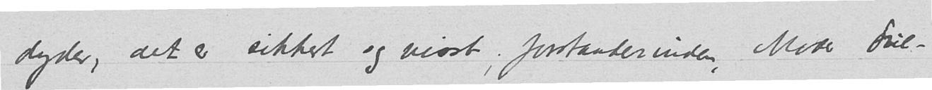dyder; det er sikkert og visst; forstanderinden, Moder Ful-
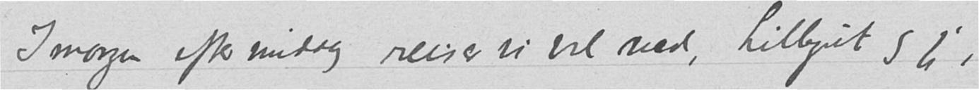Imorgen eftermiddag reiser vi vel ned, Lillegut og jeg,
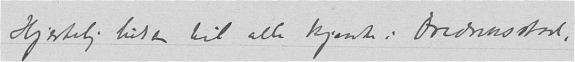Hjertelig hilsen til alle kjente i Fredriksstad,
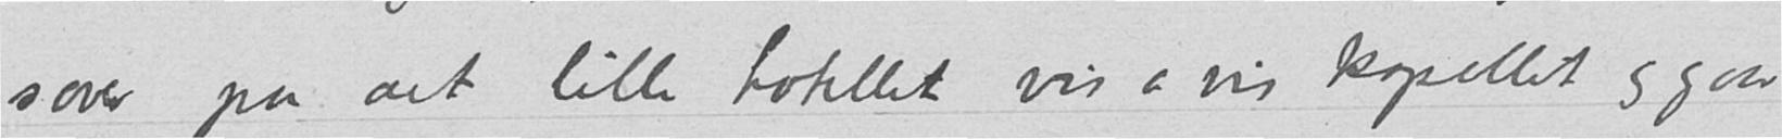sover paa det lille hotellet vis a vis kapellet og gaar
